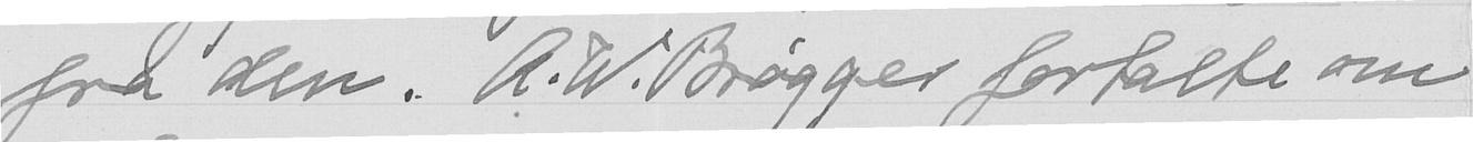fra den. A.W. Brøgger fortalte om
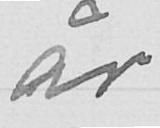år
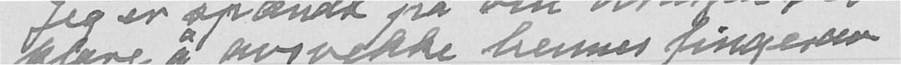klare å avsvekke hennes fingerav-
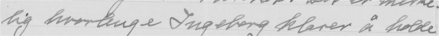lig hvorlenge Ingeborg klarer å holde
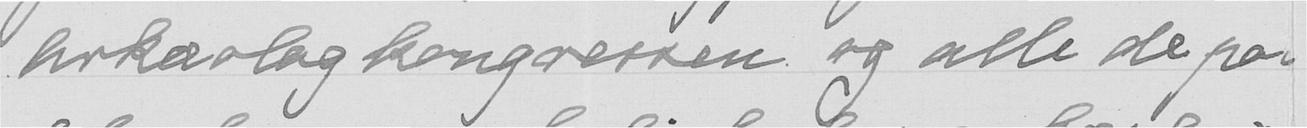arkæologkongressen og alle de po-
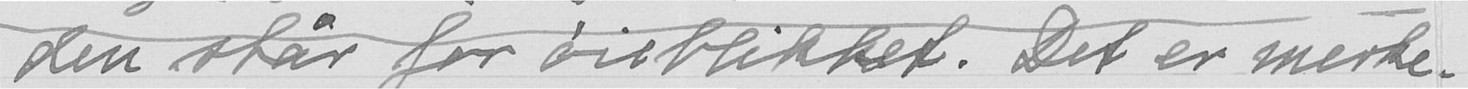den står for øieblikket. Det er merke-
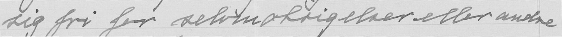sig fri for selvmotsigelser eller andre
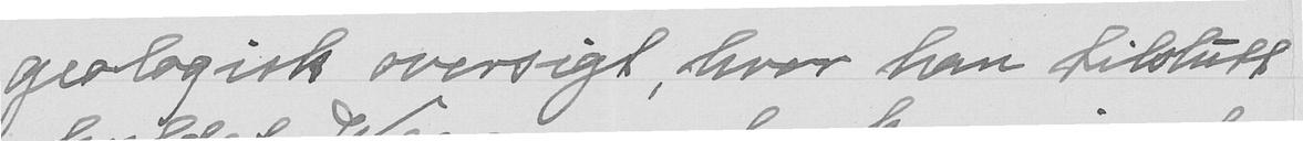geologisk oversigt, hvor han tilslutt
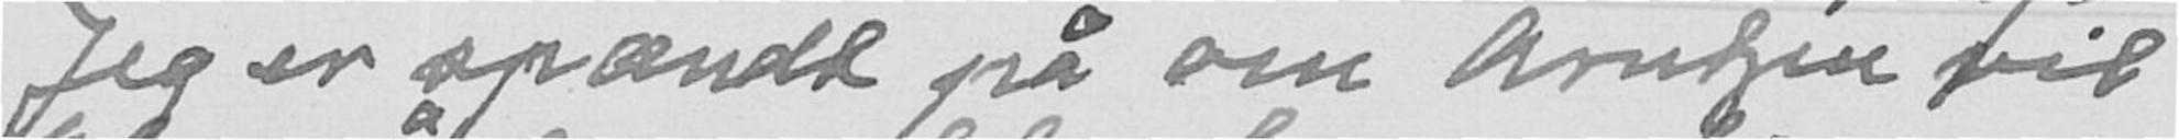Jeg er spændt på om Arntzen vil
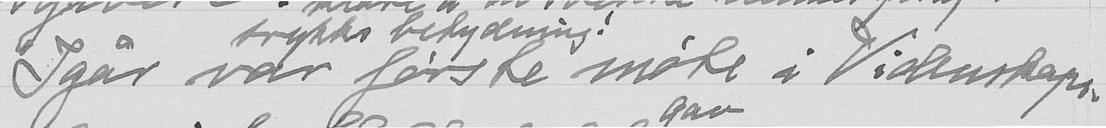I går var første møte i Videnskaps-
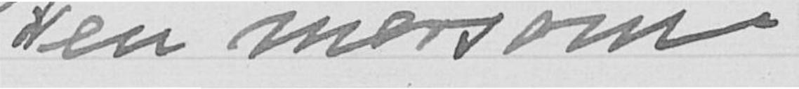en morsom
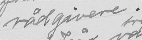rådgivere.
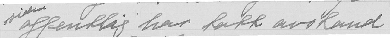offentlig har tatt avstand
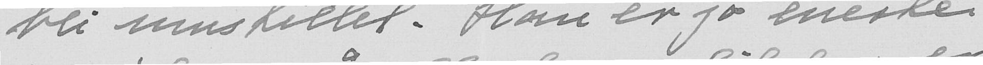bli innstillet. Han er jo eneste
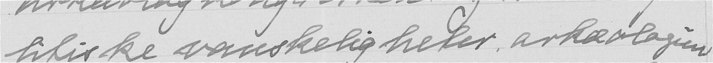litiske vanskeligheder arkæologien
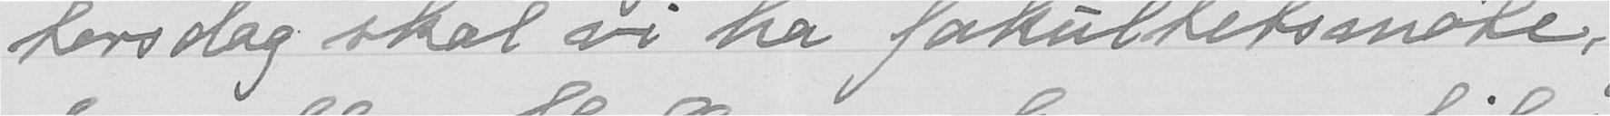torsdag skal vi ha fakultetsmøte,
